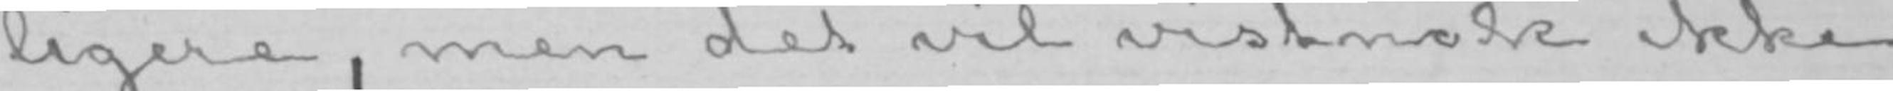ligere, men det vil vistnok ikke
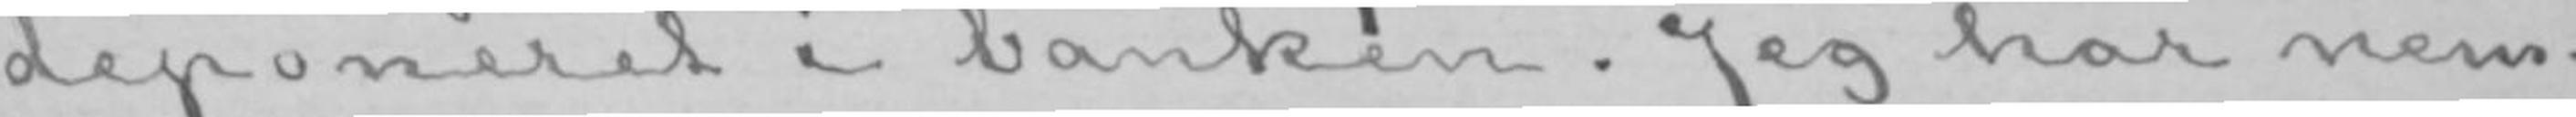deponeret i banken. Jeg har nem-
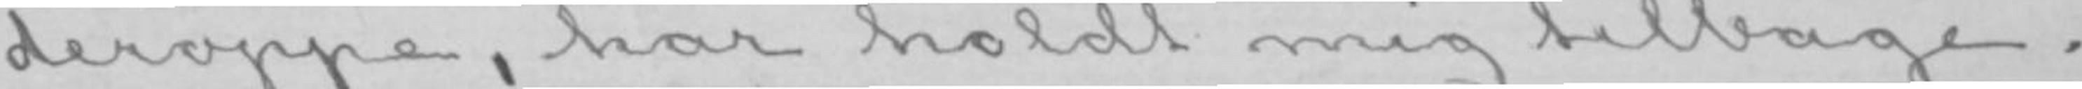deroppe, har holdt mig tilbage.
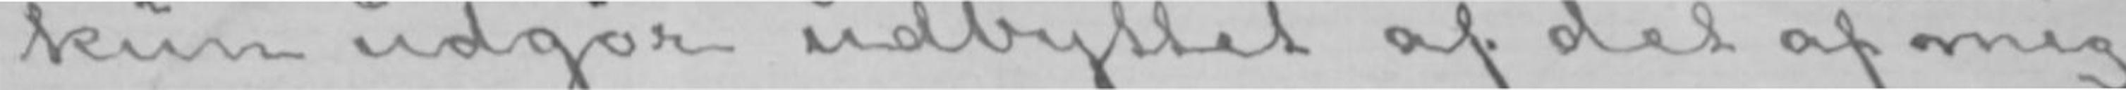kun udgør udbyttet af det af mig
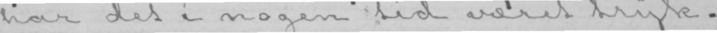har det i nogen tid været tryk-
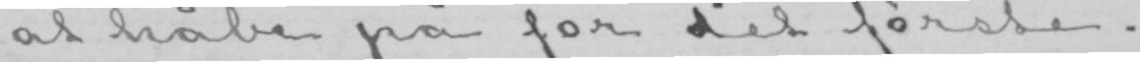at håbe på for det første.
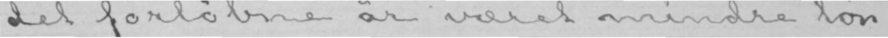det forløbne år været mindre løn-
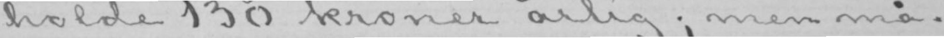holde 130 kroner årlig; men må-
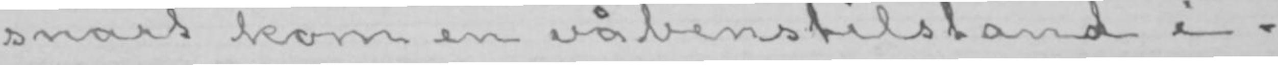snart kom en våbenstilstand i-
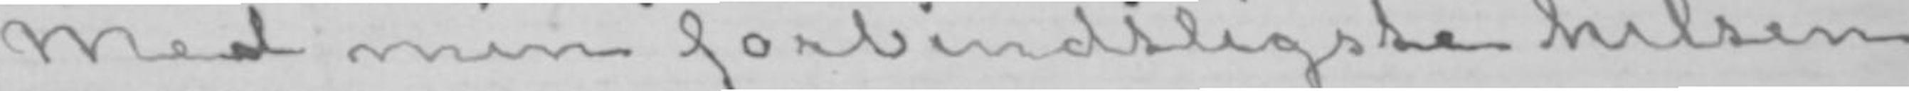Med min forbindtligste hilsen
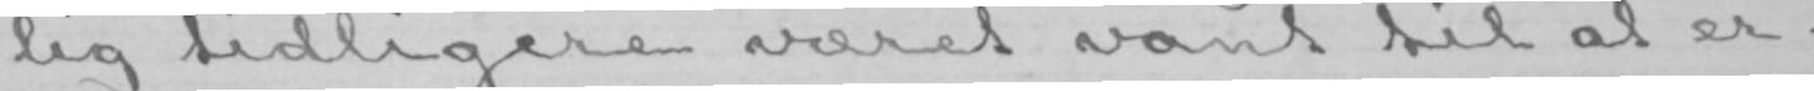lig tidligere været vant til at er-
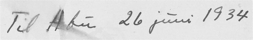Til Abu 26 juni 1934
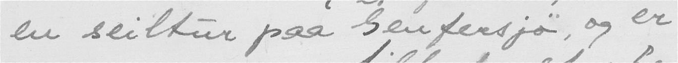en seiltur paa Benfersjø, og er
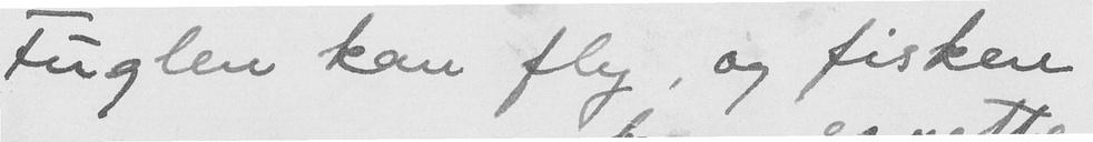Fuglen ken fly, og fisken
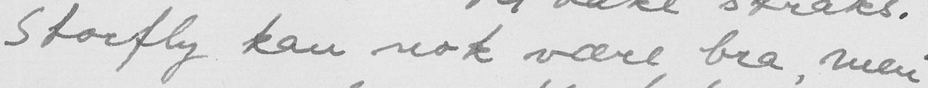storfly kan nok være bra, men
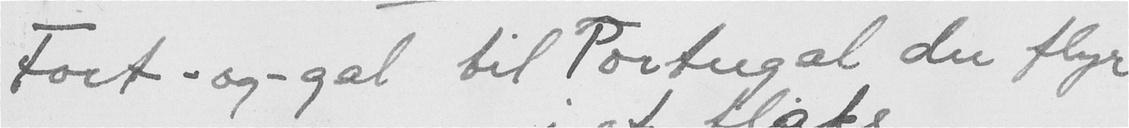Fort-og.gal til Portugal du flyr
